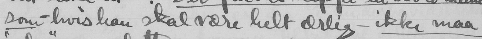som hvis han skal være helt ærlig - ikke maa
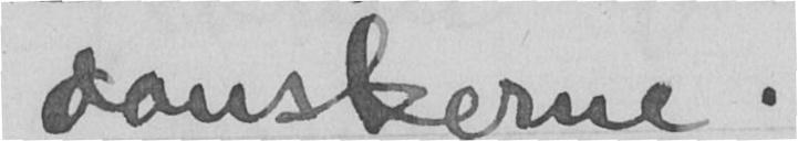danskerne.
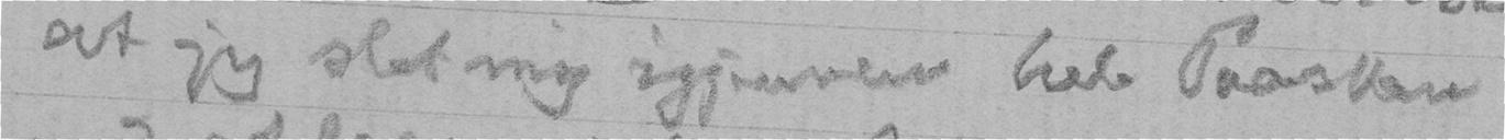at jeg slet mig igjennem hele Paasken
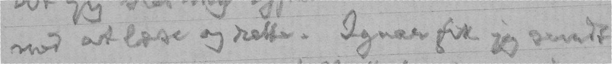med at læse og rette. Igaar fik jeg sendt
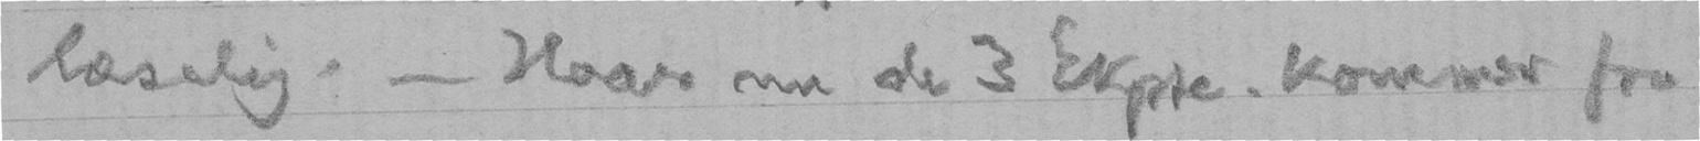læselig. - Naar nu de 3 Ekple. kommer fra
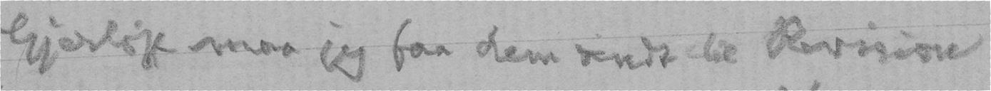Gierløff maa jeg faa dem sendt til Revision
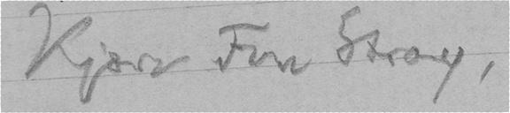Kjære Fru Stray,
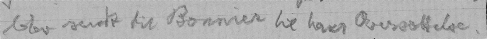blev sendt til Bonnier til hans Oversættelse.
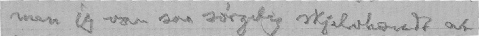men jeg var saa sørgelig skjelvhændt at
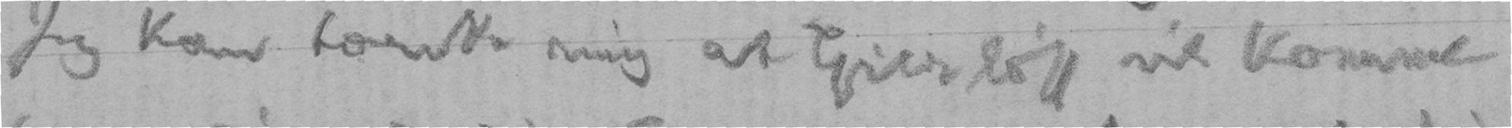Jeg kan tænke mig at Gierløff vil komme
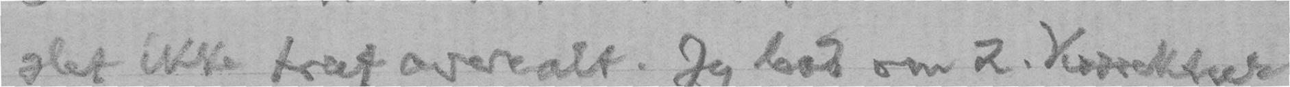slet ikke traf overalt. Jeg bad om 2. Korrektur
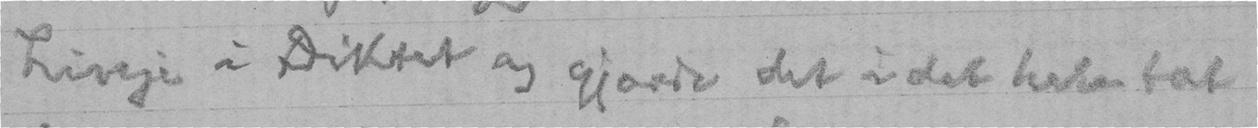Linje i Diktet og gjorde det i det hele tat
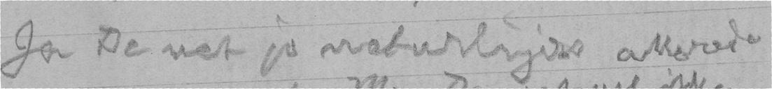Ja De vet jo naturligvis allerede
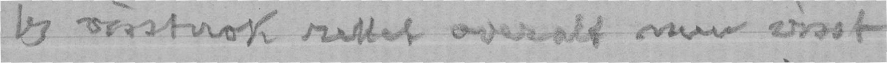jeg visstnok rettet overalt men visst
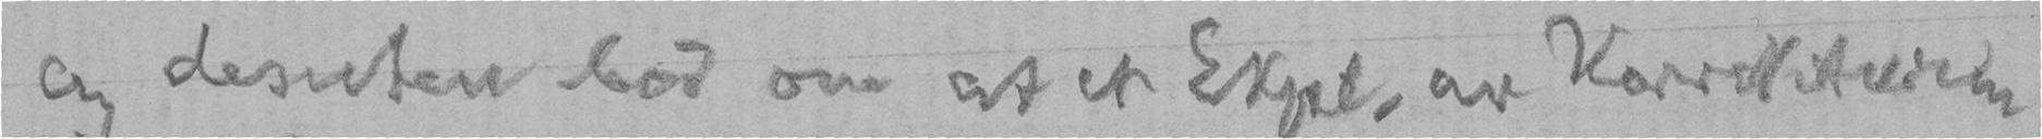og desuten bad om at et Ekpl. av Korrekturen
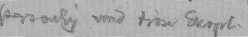personlig med disse Ekspl.
